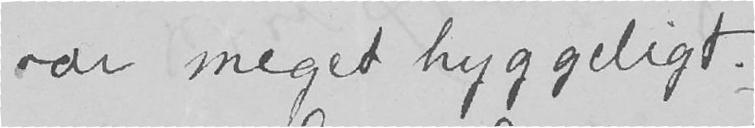var meget hyggeligt.
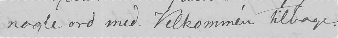nogle ord med. Velkommen tilbage.
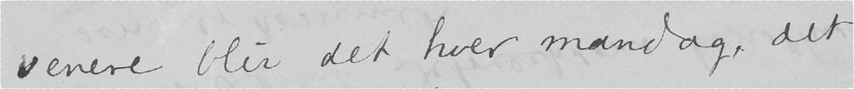senere blir det hver mandag, det
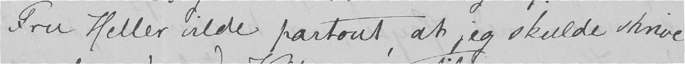Fru Heller vilde partout, at jeg skulde skrive
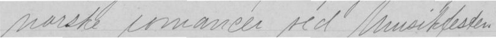norske romancer ved Musikfesten,
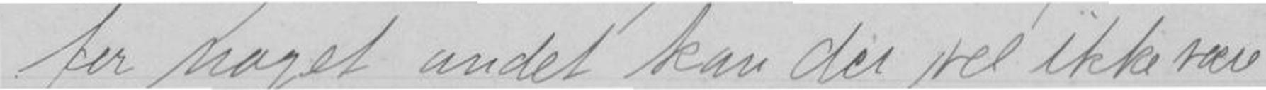for noget andet kan der vel ikke være
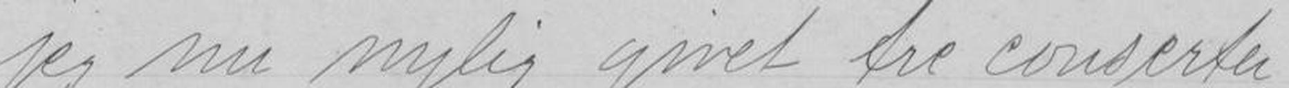jeg nu nylig givet tre conserter
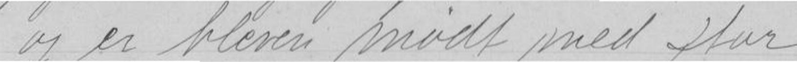og er bleven mödt med stor
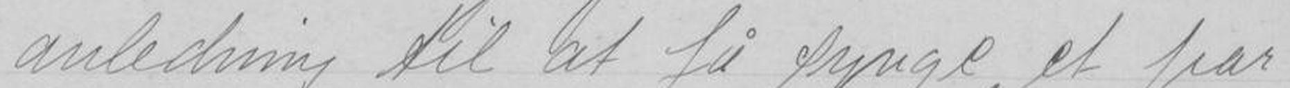anledning til at få synge et par
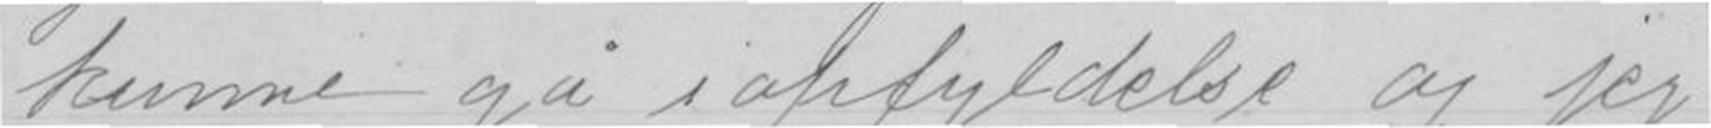kunne gå i opfyldelse og jeg
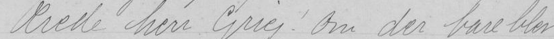Ærede herr Grieg! Om der bare blev
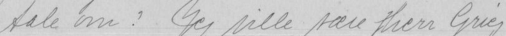tale om? Jeg ville være herr Grieg
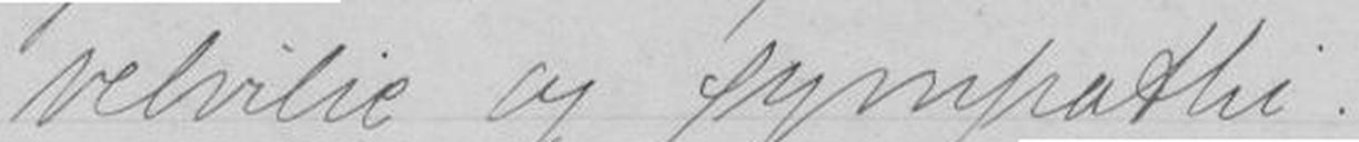velvilie og sympathi.
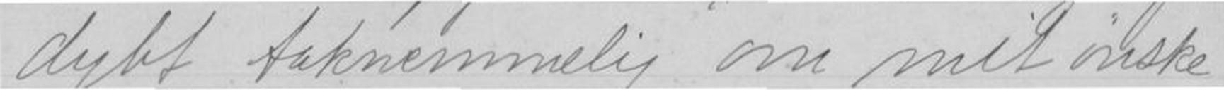dybt taknemmelig om mit önske
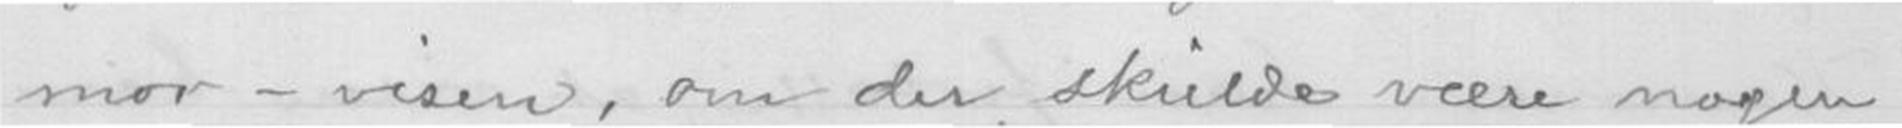mor - visen, om der skulde være nogen
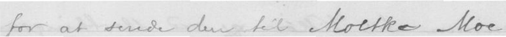for at sende den til Moltke Moe, -
